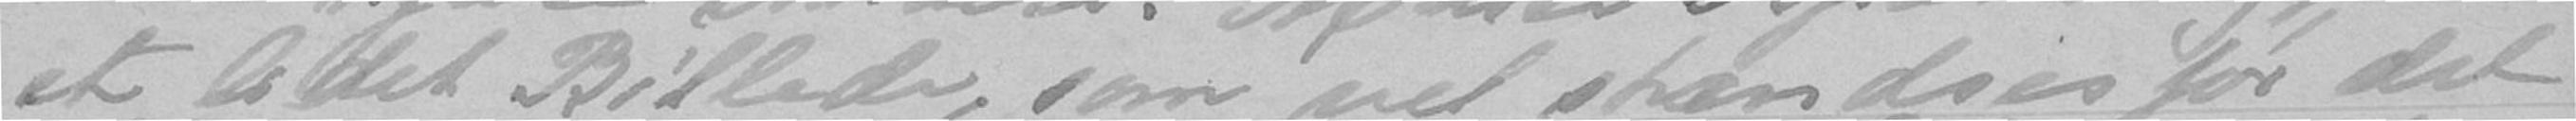et lidet Billede, som vel standses før det
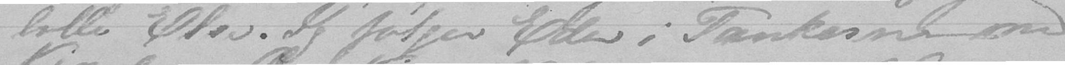lille Else. Jeg følger Eder i Tankerne med
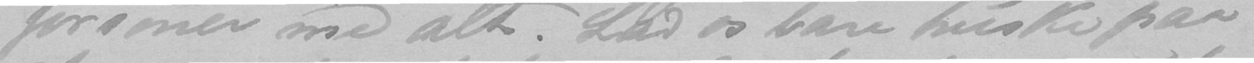forsoner med alt. Lad os bare huske paa
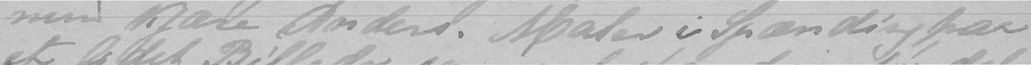min kjære Anders. Maler i Spænding paa
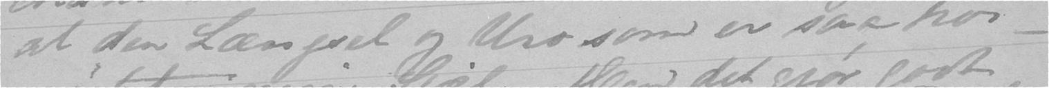at den Længsel og Uro som er saa høi-
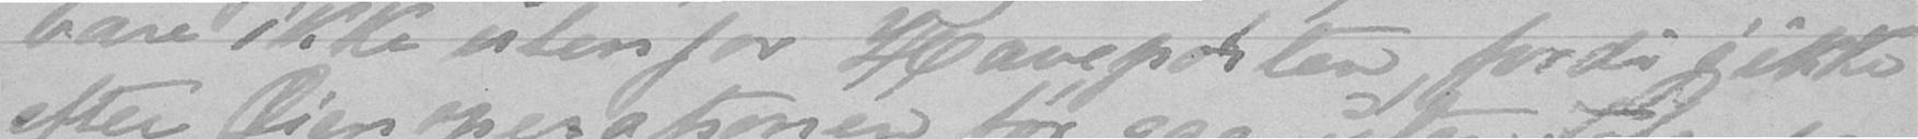bare ikke utenfor Haveporten, fordi jeg ikke
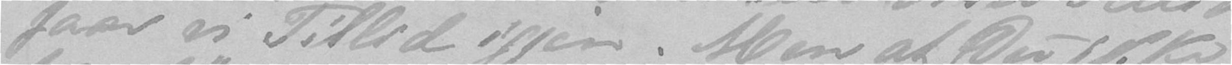faar vi Tillid igjen. Men at Du ikke
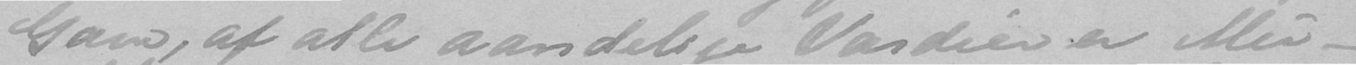Gave, af alle aandelige Værdier er Mu-
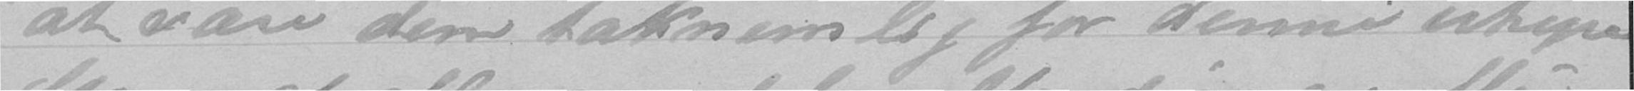at være dem taknemlig for denne uhyre
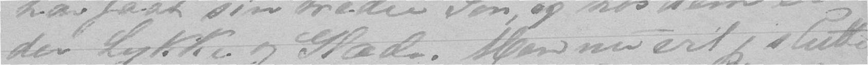der Lykke og Glæde. Men nu vil jeg slutte
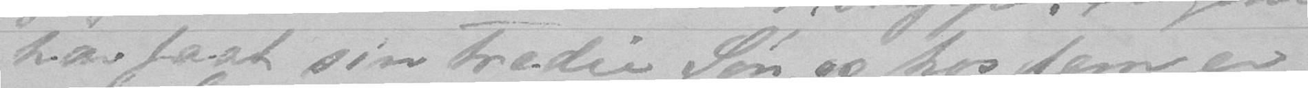har faat sin Tredie Søn, og hos dem er
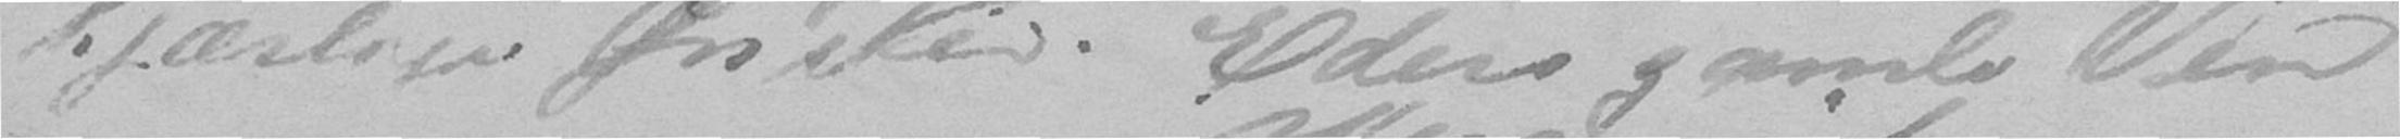Kjærlige Ønsker. Eders gamle Ven
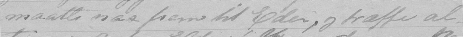maatte naa frem til Eder, og træffe al-
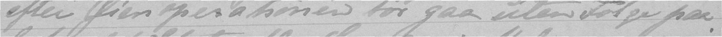efter Øienoperationen tør gaa uten Følge paa
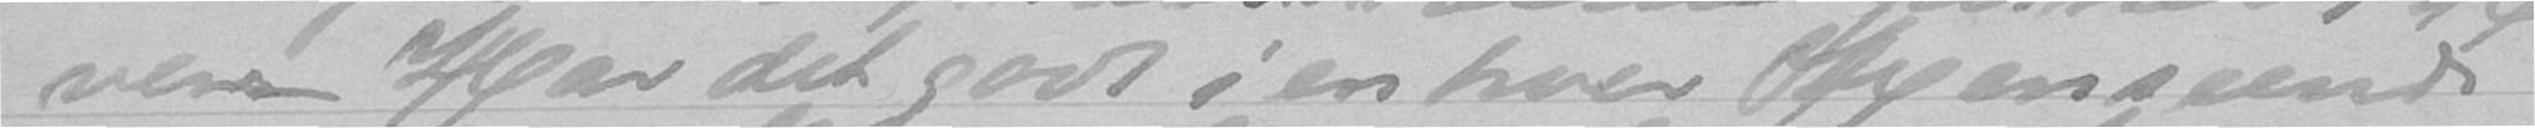ven. Har det godt i en hver Henseende
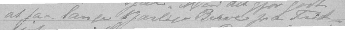at faa lange kjærlige Breve fra Frid-
